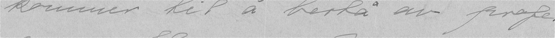kommer til å bestå av profe-
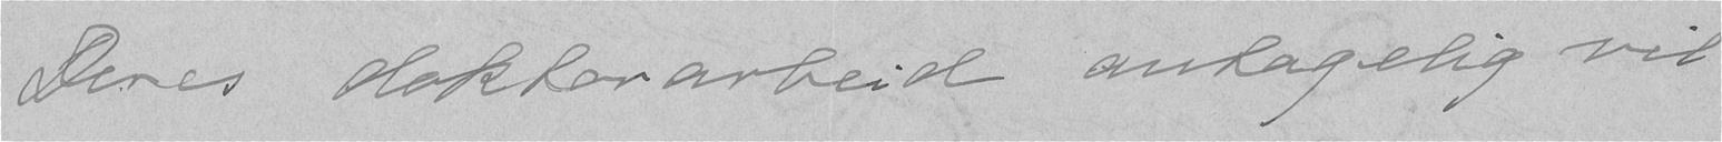Deres doktorarbeid antagelig vil
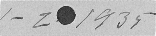1-2-1935.
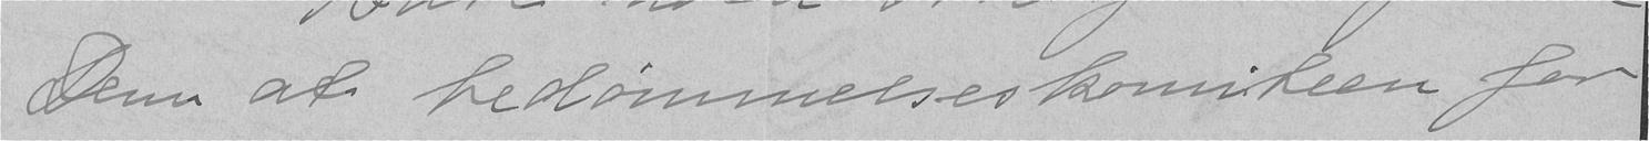Dem at bedømmelseskomiteen for
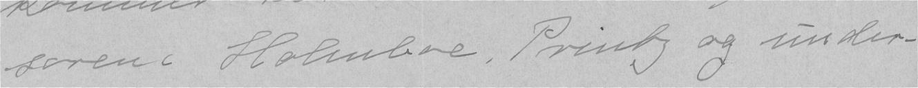sorene Holmboe, Printz og under-
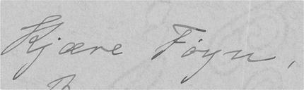Kjære Føyn,
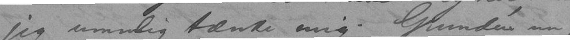jeg umulig tænke mig. Grunden nu,
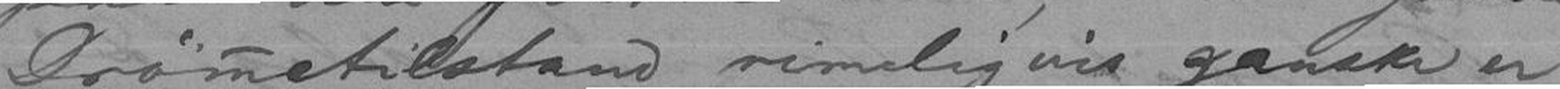Drømmetilstand rimeligvis ganske er
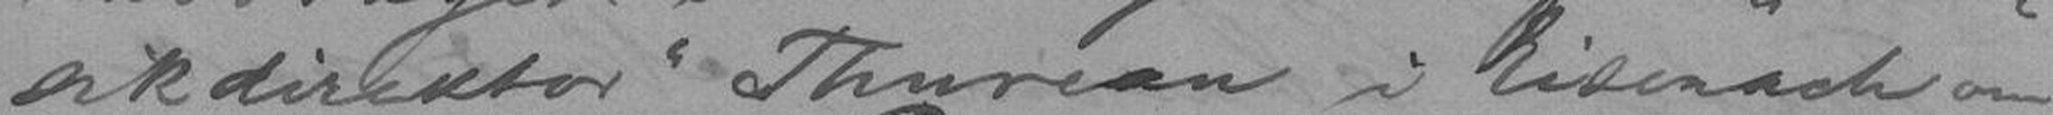sikdirektør Thureau i Eisenach om
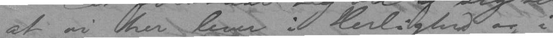at vi her lever i Herlighed og i
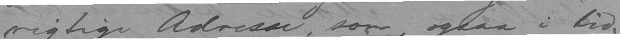rigtige Adresse, som, ogsaa i tid-
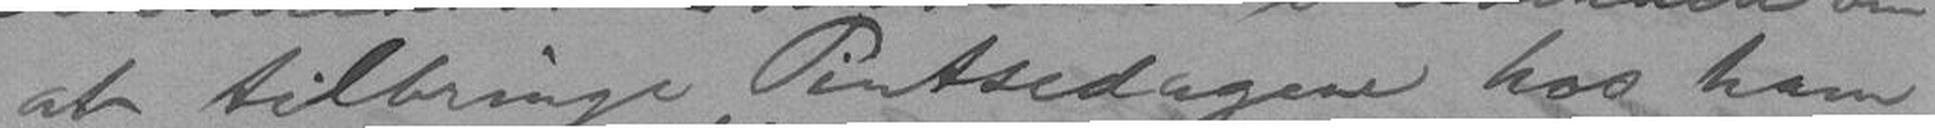at tilbringe Pintsedagene hos ham
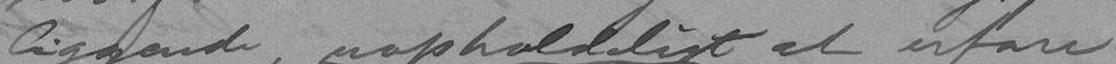liggende, uopholdeligt at erfare
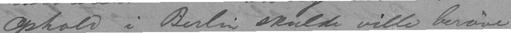Ophold i Berlin skulde ville berøve
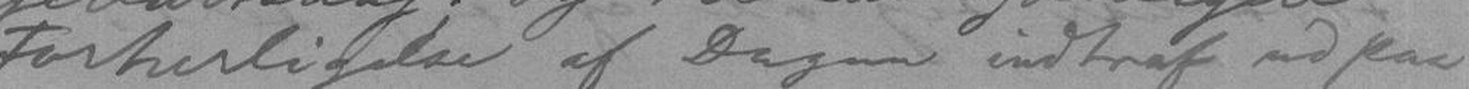Forherligelse af Dagen indtraf ud paa
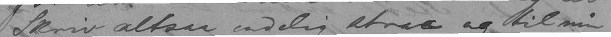Skriv altsaa endelig strax og til min
